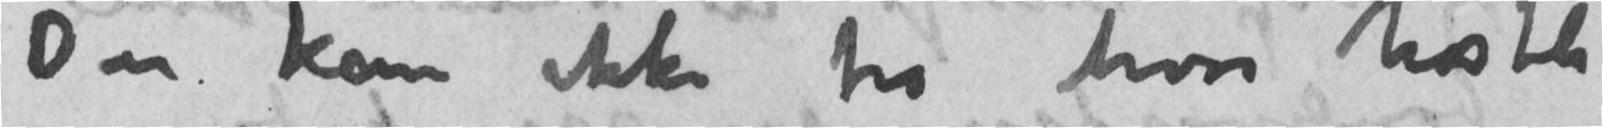Du kan ikke tro hvor høstli
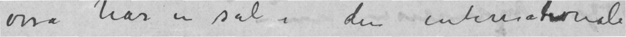osså har en sal i den internationale
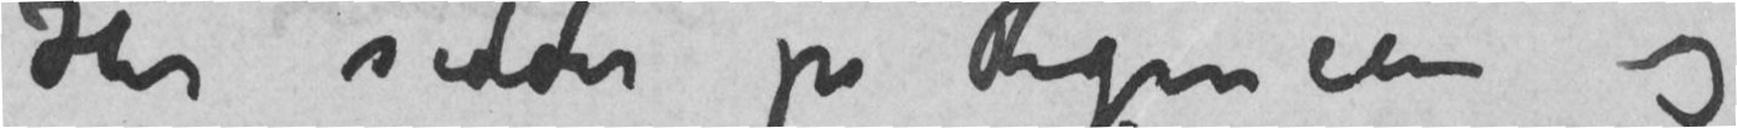Her sidder på Regence og
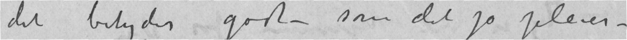det betyder godt – som det jo pleier –
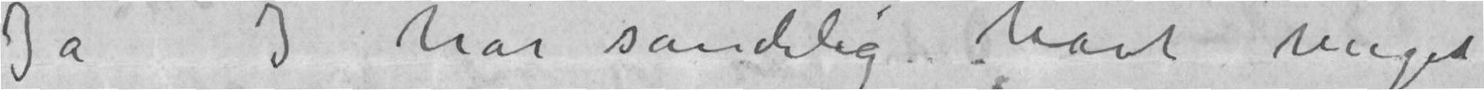Ja Jeg har sandelig havt meget
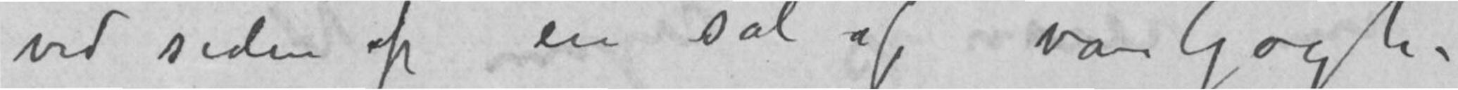ved siden af en sal af van Gogh –
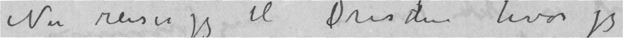Nu reiser jeg til Dresden hvor jeg
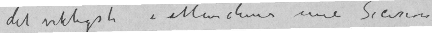det viktigste i MünchensSecesion
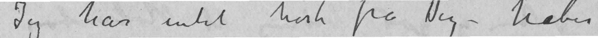Jeg har intet hørt fra Dig – håber
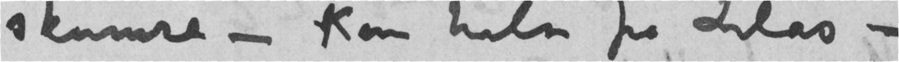skumre – Kan hilse fra Lilas –
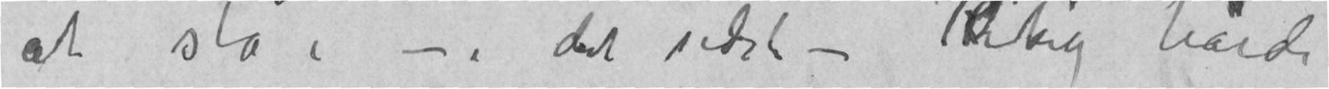at stå i– det sidste – Riktig hårde
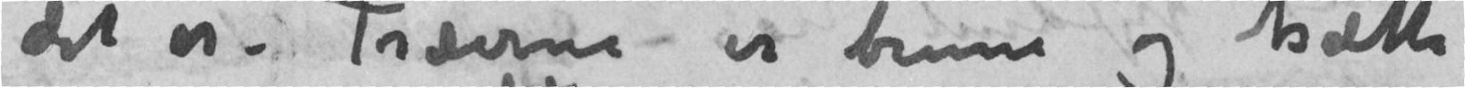det er – Træerne er brune og trætte
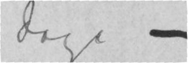dage –
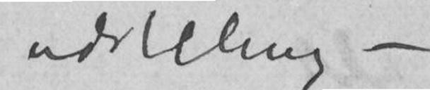udstilling –
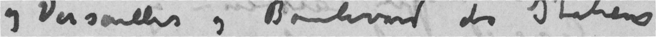og Versailles og Boulevard des Italiens
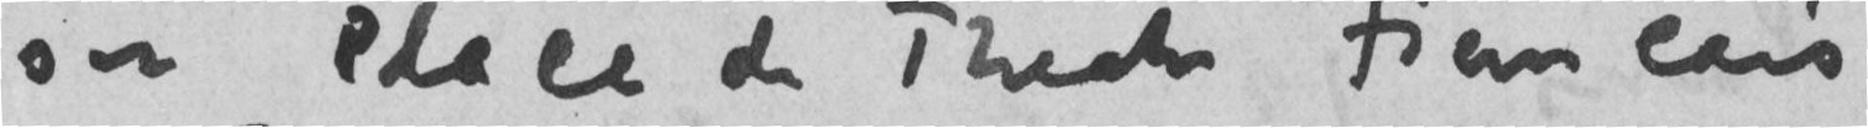ser Place de Theatre Francais
